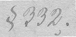§ 332.
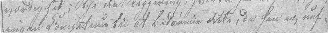ingen Competence til at bedømme dette, da han er uaf-
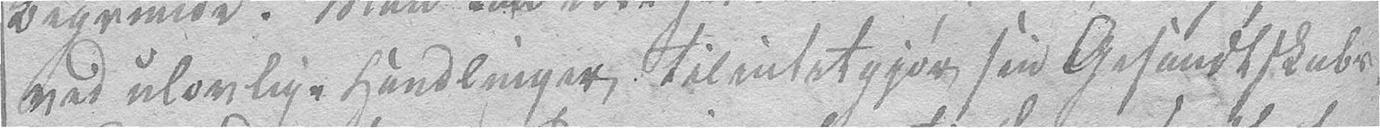ved ulovlige Handlinger tilintetgjør sin Gesandtskabs-
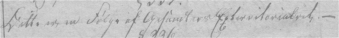Dette er en Følge af Gesandteres Exterritorialitet.–
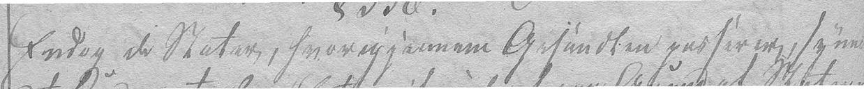Endog de Stater, hvorigjennem Gesandten passerer, synes
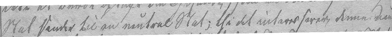Stat sender til en neutral Stat; thi det interesserer denne neu-
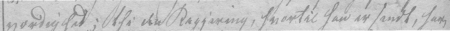værdighed; thi den Regjering, hvortil han er sendt, har
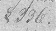§ 336.
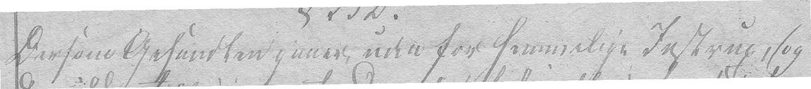Dersom Gesandten gaaer udenfor hemmelige Instrux, og
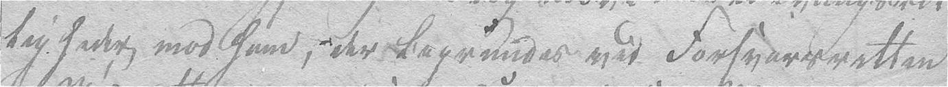tigheder mod ham, der begrundes ved Forsvarsretten
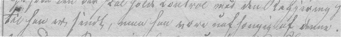til han er sendt, maa han være uafhængig af denne.
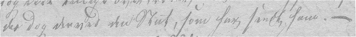des dog derved den Stat, som har sendt ham. –
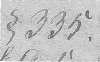§ 335.
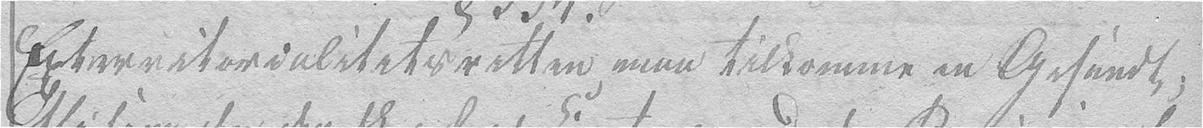Exterritorialitetsretten maa tilkomme en Gesandt;
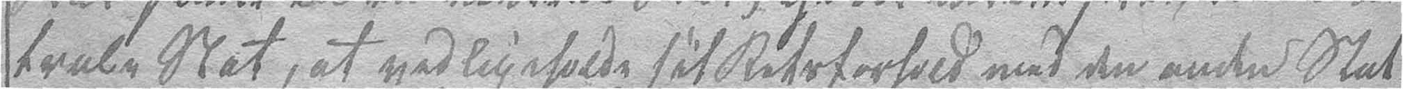trale Stat, at vedligeholde sit Retsforhold med den anden Stat,
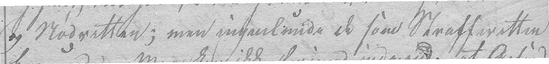og Nødretten; men ingenlunde de som Strafferetten
